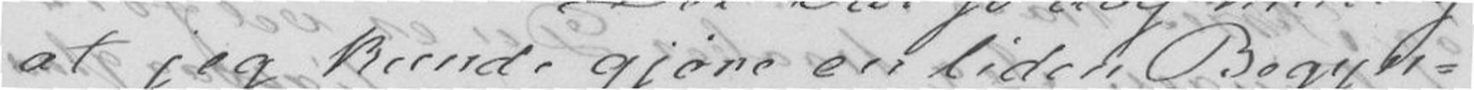at jeg kunde gjøre en liden Begyn-
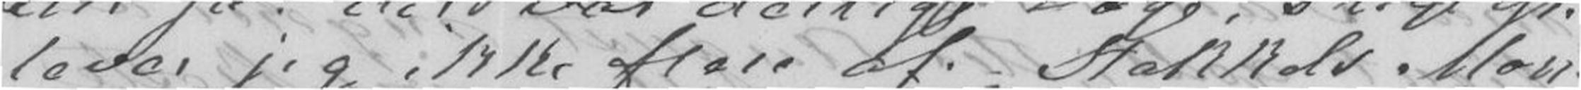lever jeg ikke flere af. Stakkels Moer
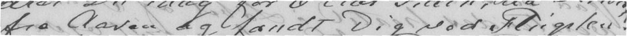fra Aasen og fandt Dig ved Flügelen.
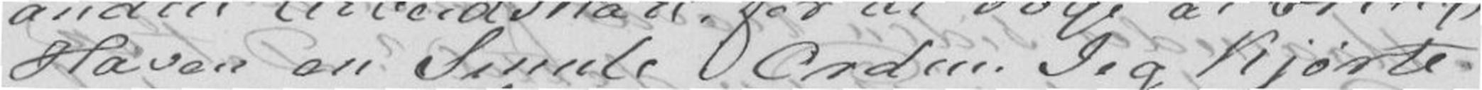Haven en Smule i Orden. Jeg kjørte
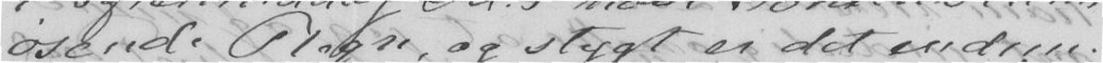øsende Regn, og stygt er det endnu.
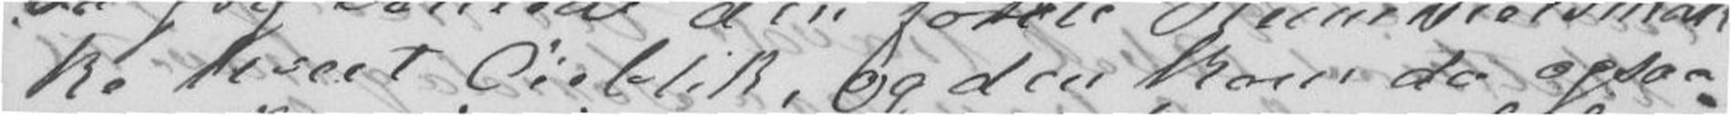ke hvert Øieblik, og den kom da ogsaa
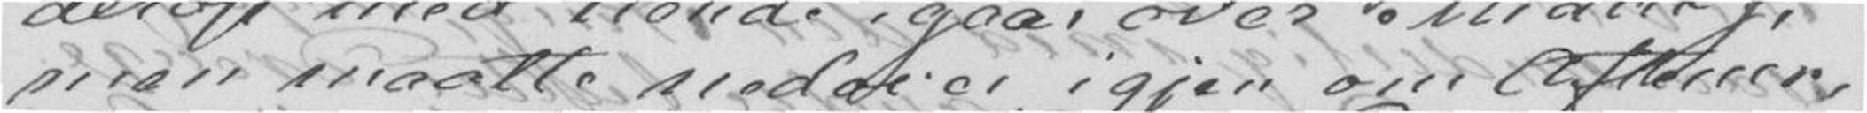men maatte nedover igjen om Aftenen
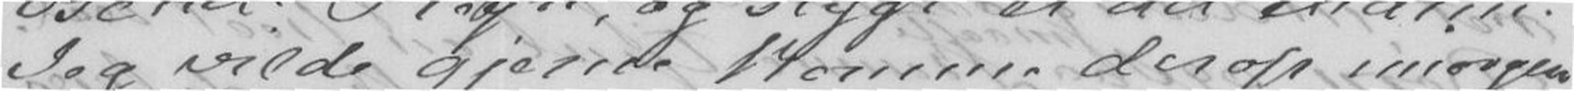Jeg vilde gjerne komme derop imorgen,
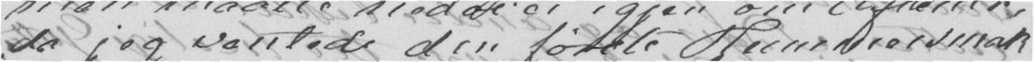da jeg ventede den første Hummersmak-
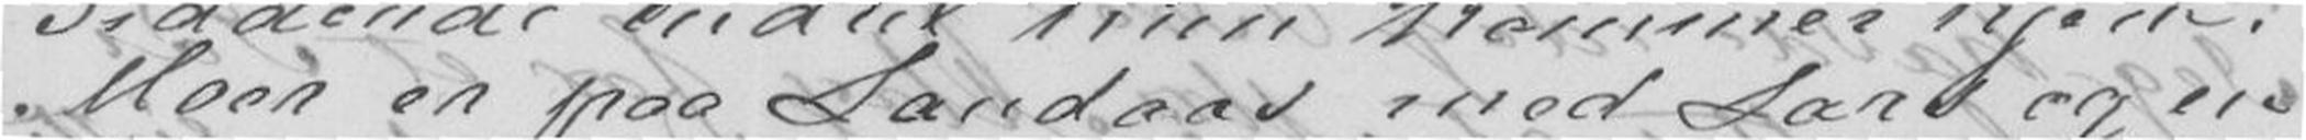Moer er paa Landaas med Lars og en
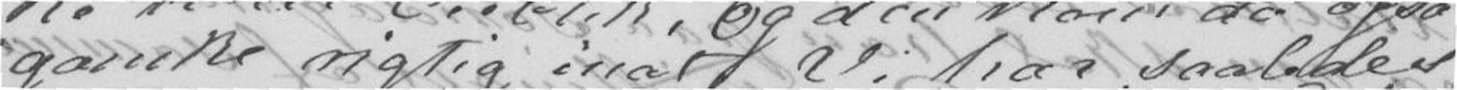ganske rigtig inat. Vi har saaledes
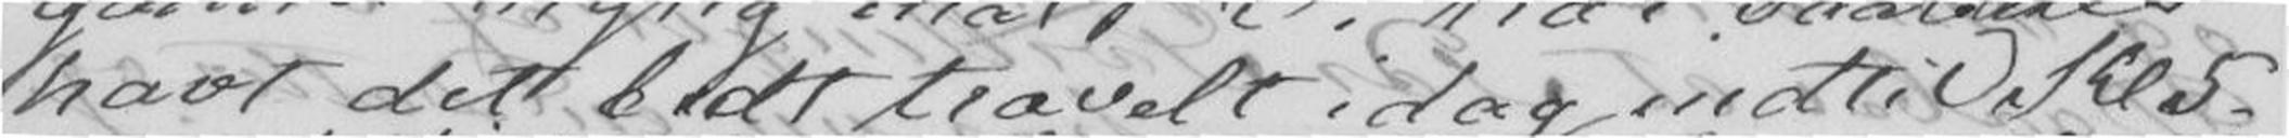havt det lidt travelt idag indtil Kl 5.
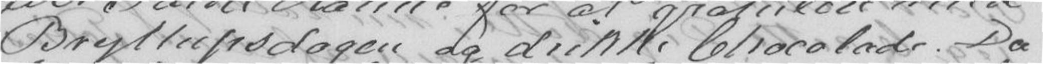Bryllupsdagen og drikke Chocolade. Da
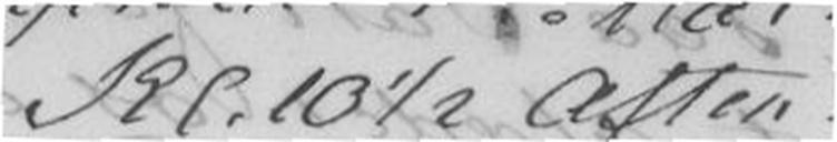Kl.10½ Aften.
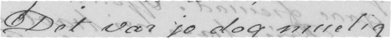Det var jo dog muelig
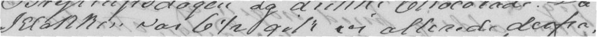Klokken var 6½ gik vi allerede derfra,
